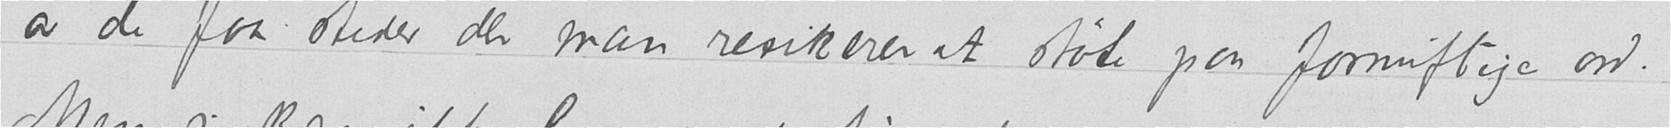av de faa steder der man risikerer at støte paa fornuftige ord.
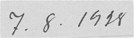7.8.1928
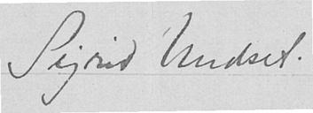Sigrid Undset.
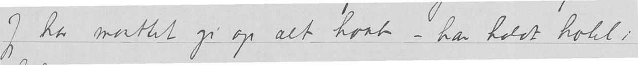Jeg har maattet gi op alt haab – har holdt hotel i
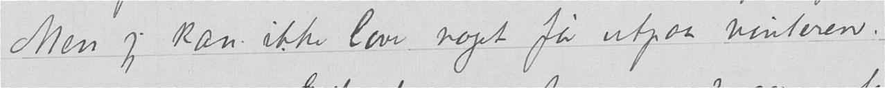Men jeg kan ikke lave noget før utpaa vinteren.
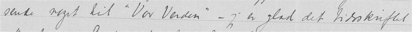sende noget til «Vor Verden» – jeg er glad det tidsskriftet
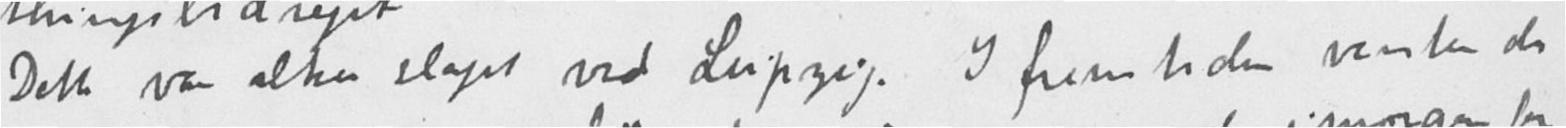Dette var altsaa slaget ved Leipzig. I fremtiden venter de
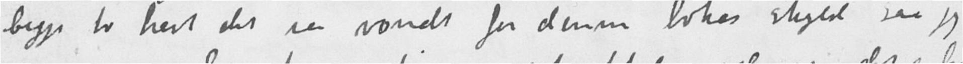begge to havt det saa vondt for denne bokens skyld saa jeg
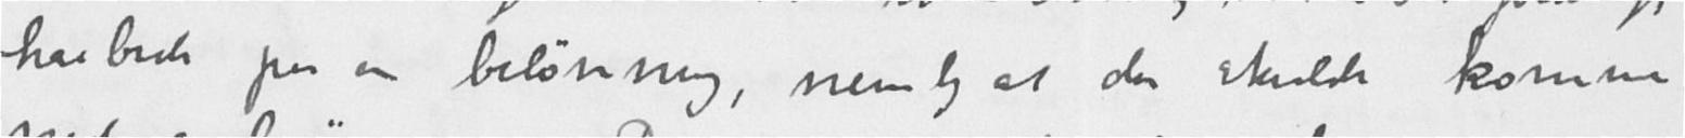haabede paa en belønning, nemlig at du skulde komme
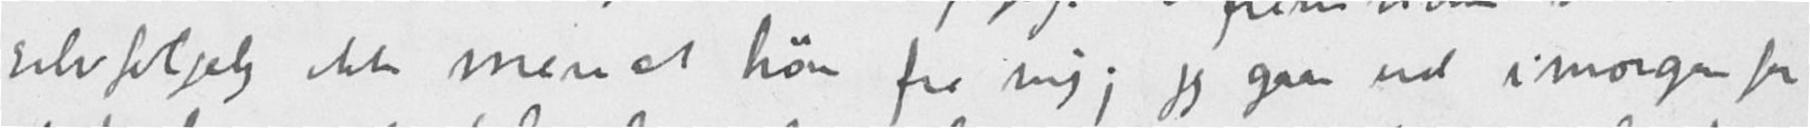selvfølgelig ikke mere at høre fra sig; jeg gaar ud i morgen for
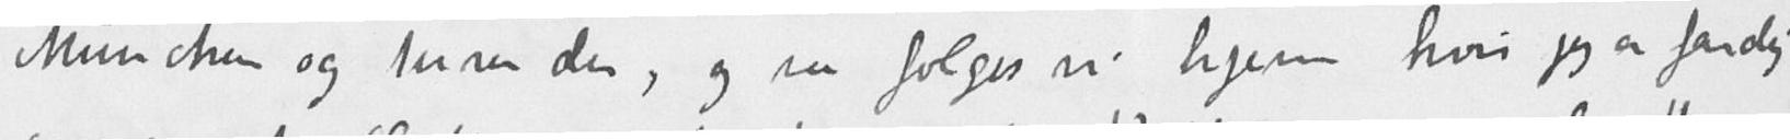München og turer der, og saa følges vi hjem hvis jeg er færdig
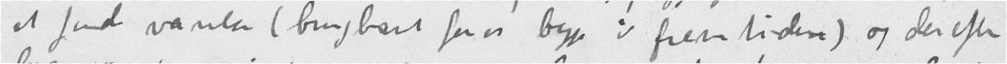at finde værelse (brugbart for os begge i fremtiden) og derefter
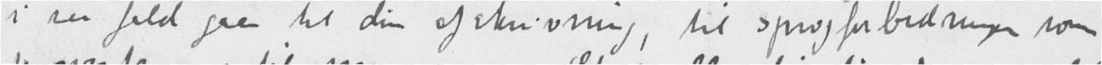i saa fald gaar til din afskrivning, til sprogforbedringer som
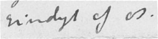sindigt af os.
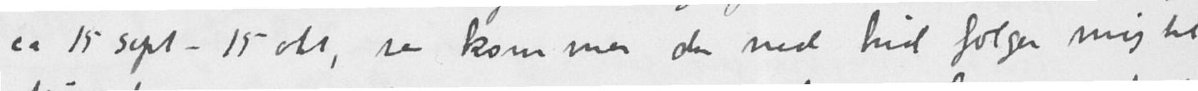ca 15 sept - 15 okt, saa kommer du ned hid følger mig til
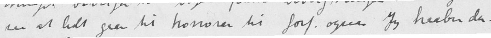saa at lidt gaar til honorar til forf. ogsaa. Jeg haaber der-
